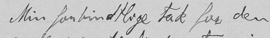Min forbindtlige tak for den
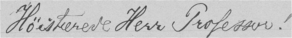Høistærede Herr Professor!
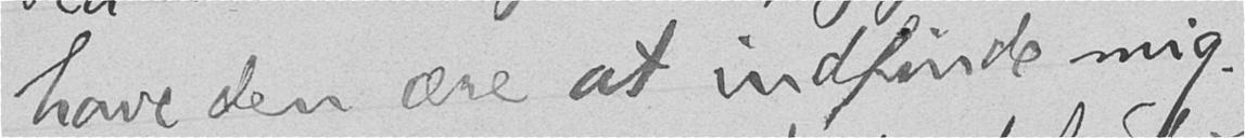have den ære at indfinde mig.
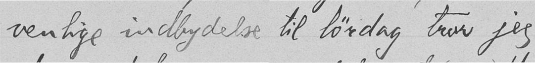venlige indbydelse til lørdag tror jeg
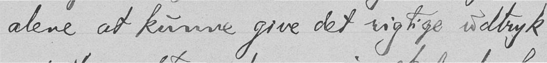alene at kunne give det rigtige udtryk
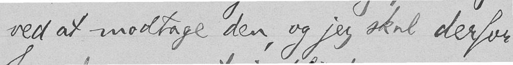ved at modtage den, og jeg skal derfor
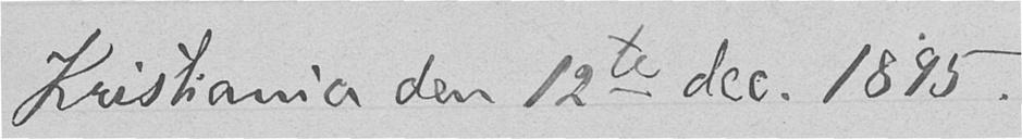Kristiania den 12te dec. 1895.
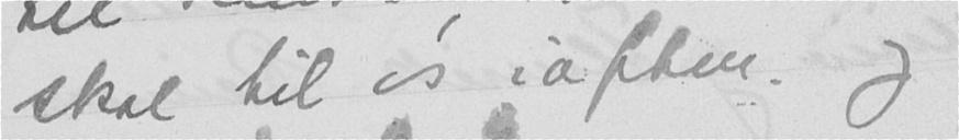skal til os iaften. og
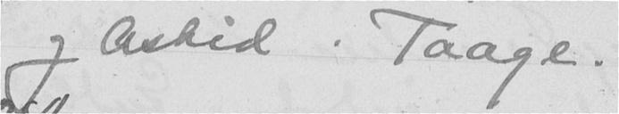og Astrid. Taage.
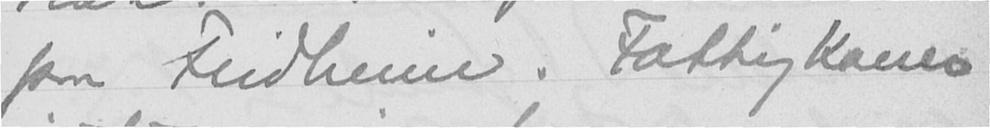paa Fridheim. Fattigkoner
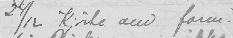24/12 Kjørte om form.
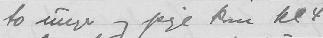to unger og pige kom kl 4
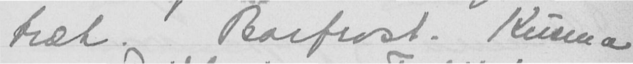træt. Barfrost. Krisen er
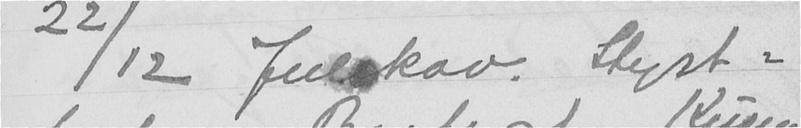22/12 Julekav. Styrt-
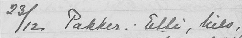23/12 Pakker. Etti, Nils,
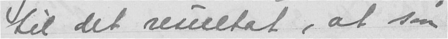til det resultat, at saa
